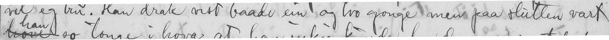vil eg tru. Han drak vist baade ein og tvo gonge men paa slutten vart
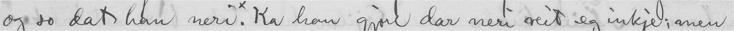og so dat han neri. Ka han gjore dar neri veit eg inkje; men
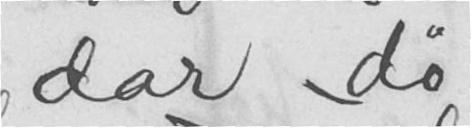dar dø
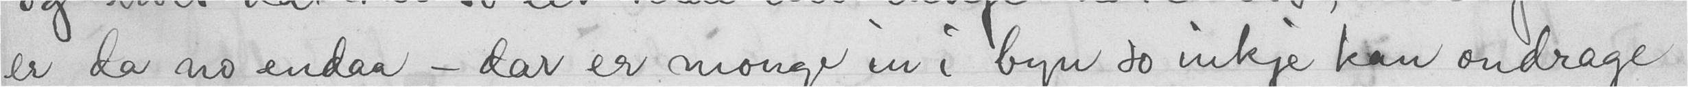er da no endaa - dar er monge in i byn so inkje kan ondrage
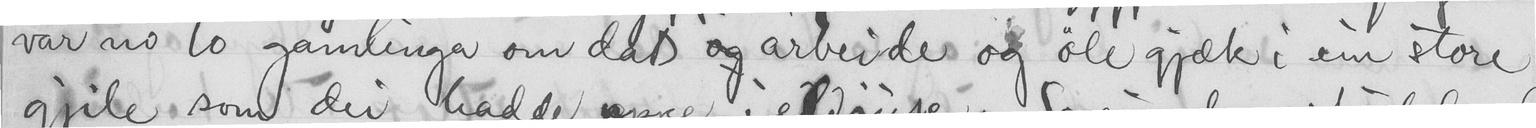var no to gamlinga om dat og arbeide og øle gjæk i ein store
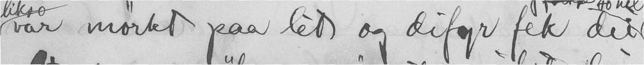var mørkt paa lit og difyr fek dei
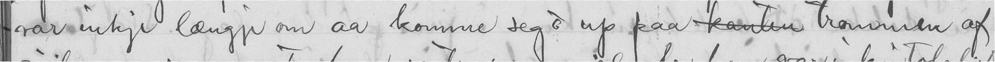var inkje længje om aa komme seg  up paa  trommen, af
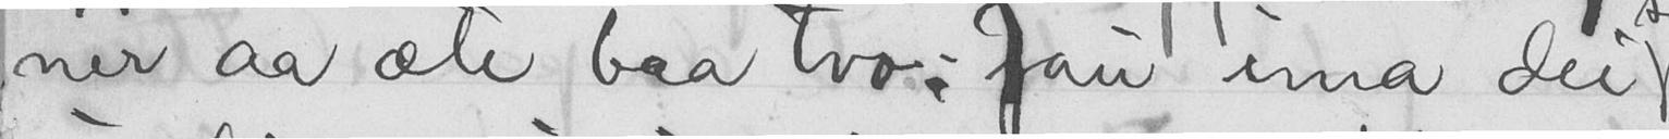ner aa æte baa tvo: Jau inna dei
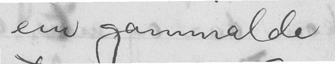ein gammalde
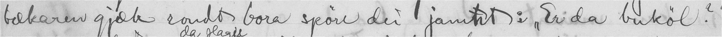bækaren gjæk rondt bora spøre dei jamt: "Er da bukøl?"
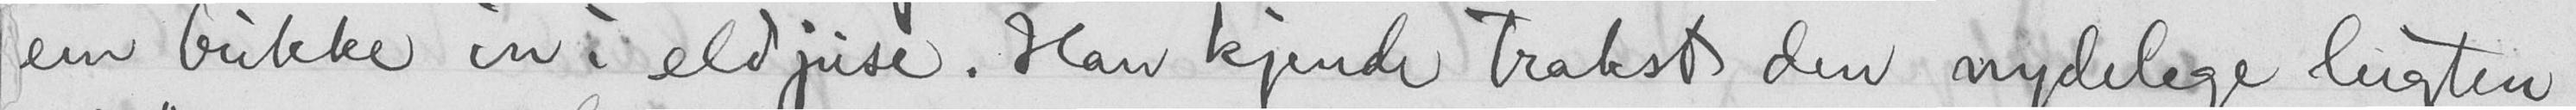ein bukke in i eldjuse. Han kjende trakst den nydelige lugten
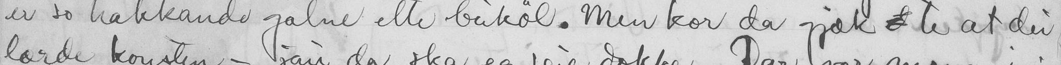er so hakkande galne elle bukøl. Men kor da gjæk  te at dei
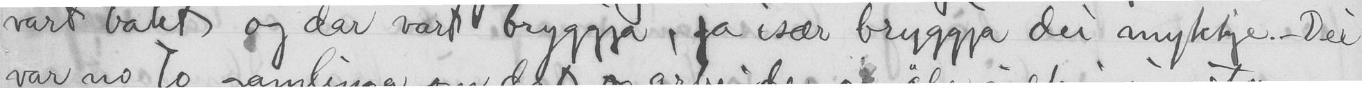vart bakt og dar vart bryggja, ja især bryggja dei myktje. - Dei
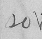so
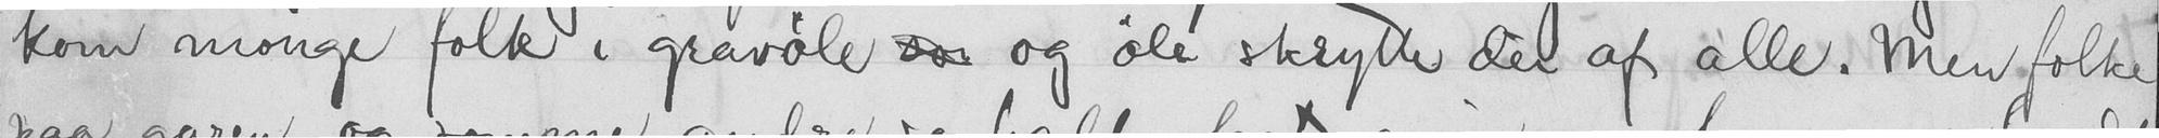kom monge folk i gravøle  og øle skrytte dei af alle. Men folke
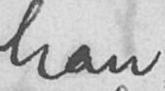han
